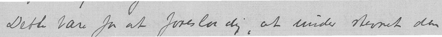Dette bare for at foreslaa dig, at under stevnet den
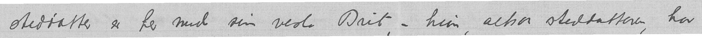steddatter er her med sin vesle Brit, – hun, altsaa steddatteren, har
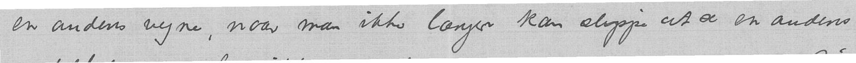en andens vegne, naar man ikke længer kan slippe at se en andens
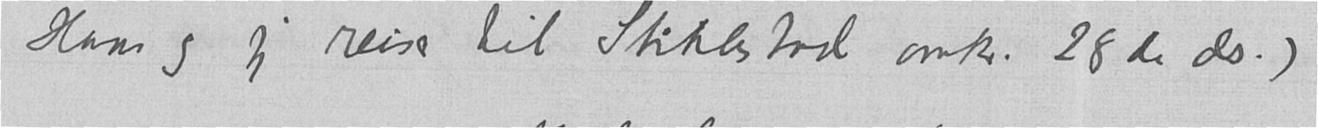Hans og jeg reiser til Stiklestad omkr. 28de ds.)
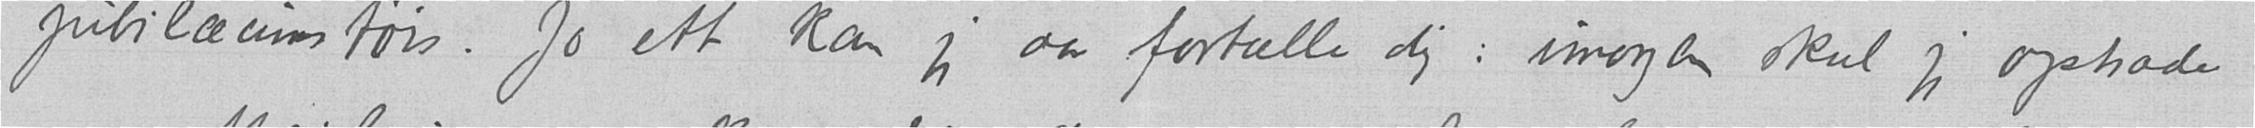jubilæumstøis. Jo ett kan jeg da fortælle dig: imorgen skal jeg optræde
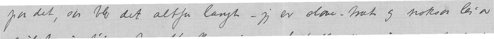paa det, saa blev det altfor langt – jeg er slave-træt og noksaa lei av
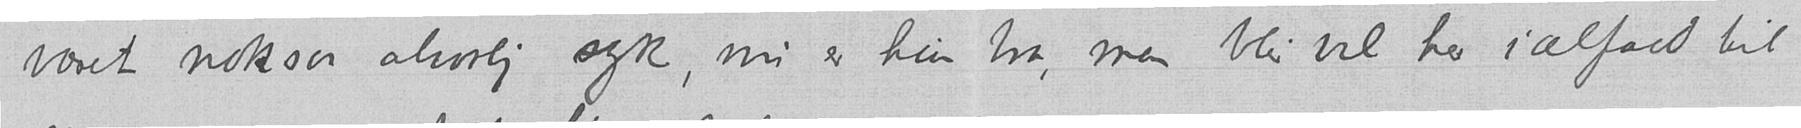været noksaa alvorlig syk, nu er hun bra, men blir vel her ialfald til
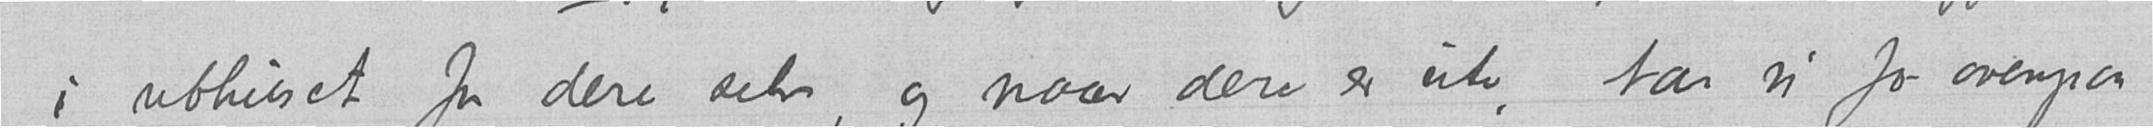i uthuset for dere selv, og naar dere er ute, tar vi jo ovenpaa
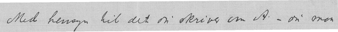Med hensyn til det du skriver om A. – du maa
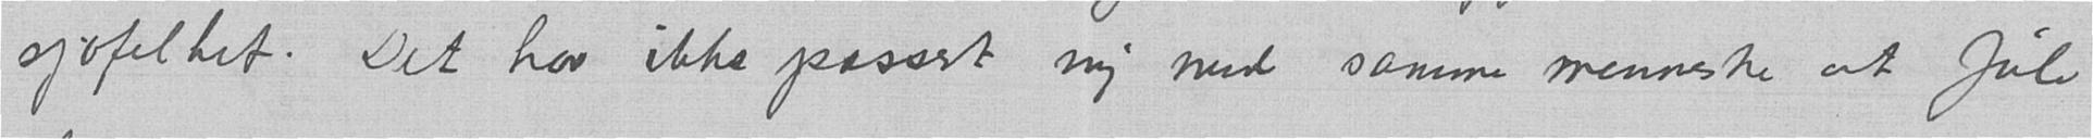sjofelhet. Det har ikke passert mig med samme menneske at føle
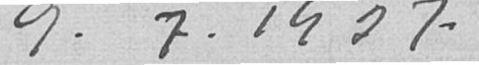9.7.1927.
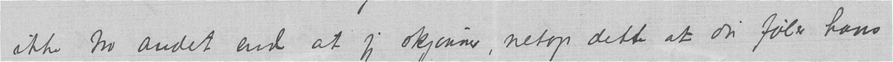ikke tro andet end at jeg skjønner, netop dette at du føler hans
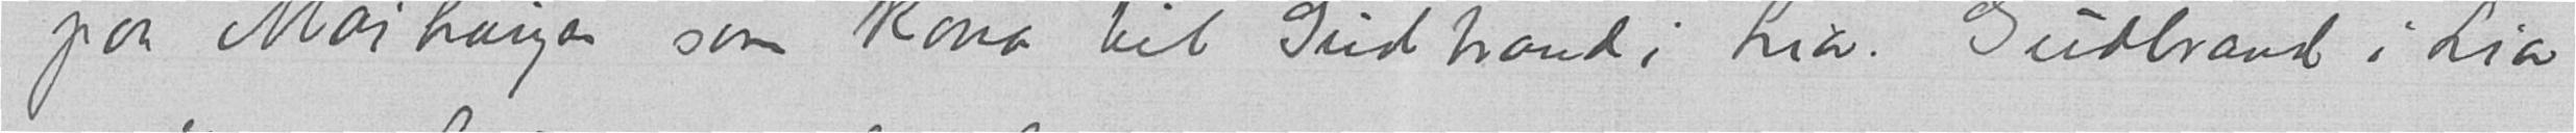paa Maihaugen som kona til Gudbrand i Lia. Gudbrand i Lia
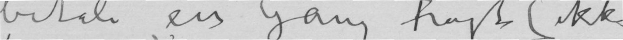betale en Gang Fragt (ikke
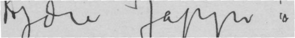Kjære Jappe!
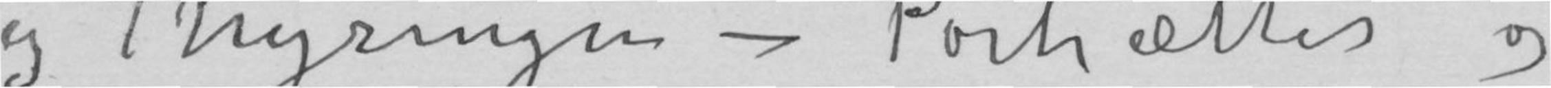og Thyringen – Portrætter
